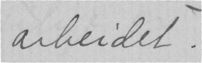arbeidet.
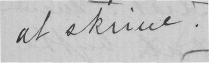at skrive.
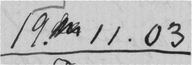19.11.03
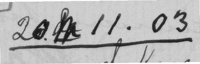20.  11.02
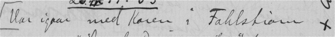Var igaar med Karen i Fahlstrøm x
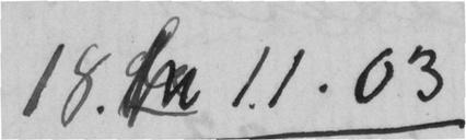18.  11.03
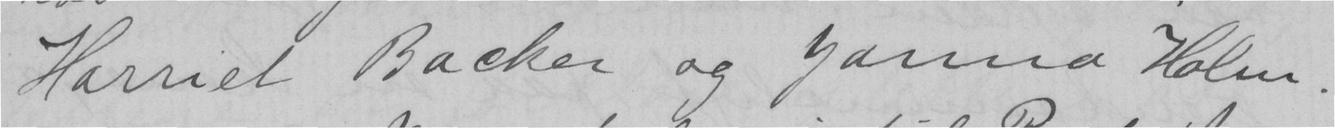Harriet Backer og Janna Holm.
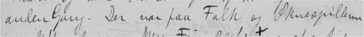anden Gang. Der var faa Folk og Skuespillern
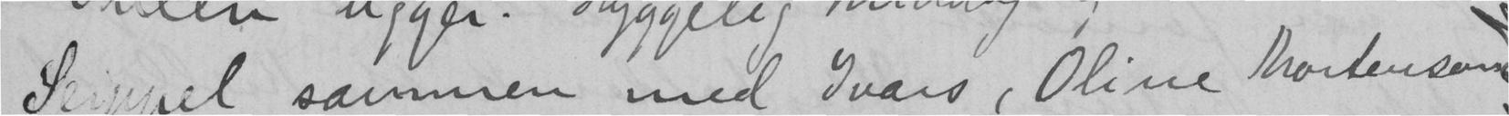Seippel sammen med Ivars, Oline Mortenson
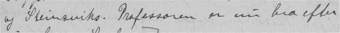og Steinsviks. Professoren er nu bra efter
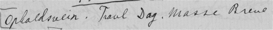Ophaldsveir. Travl Dag. Masse Breve
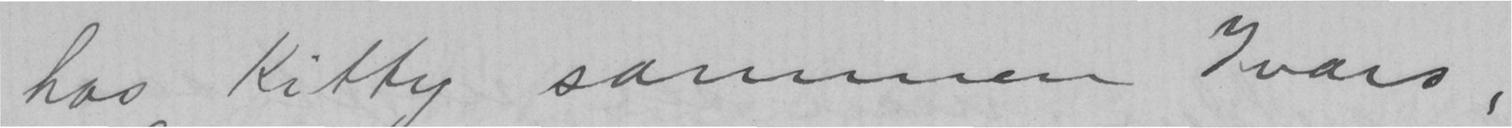hos Kitty sammen Ivars,
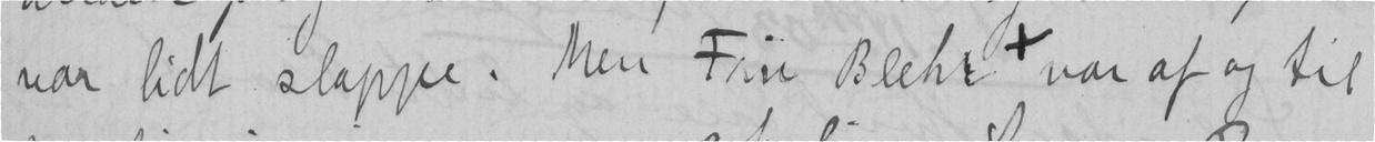var lidt slappe. Men Fru Bleh var af og til
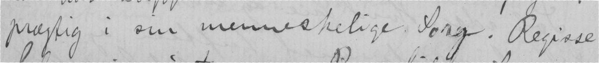prægtig i sin menneskelig Sorg. Regisse
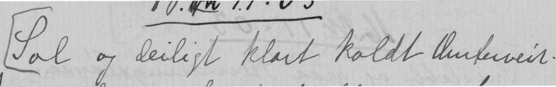Sol og deiligt klart koldt vinterveir.
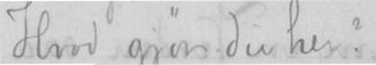Hvad gjør du her?
